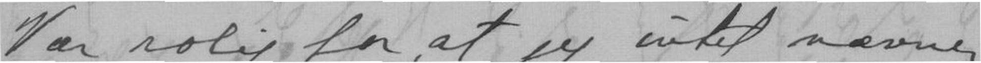Var rolig for, at jeg intet nævner
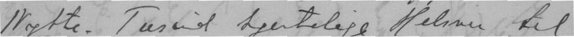Nytte. Tusind hjertelige Hilsner til
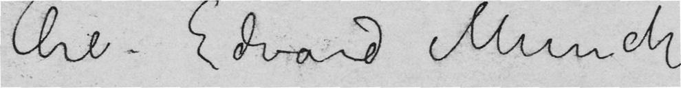Ærb. Edvard Munch
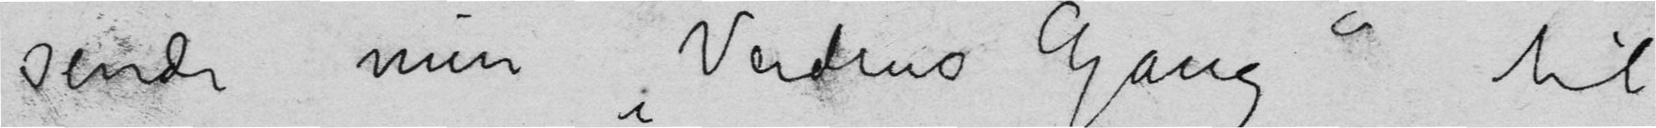sende min «Verdens Gang» til
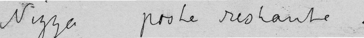Nizza poste restante,
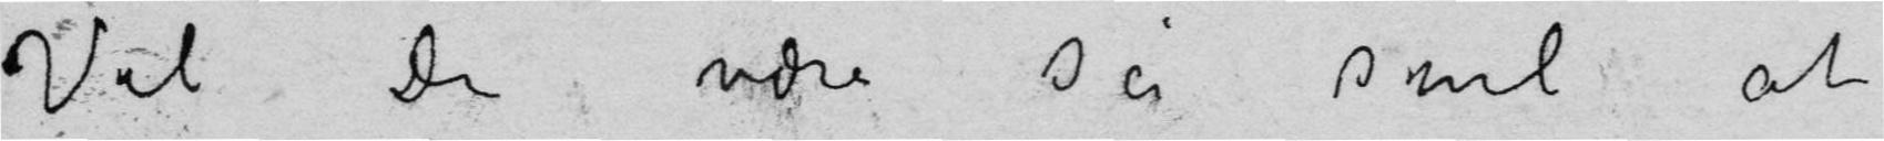Vil De være så snil at
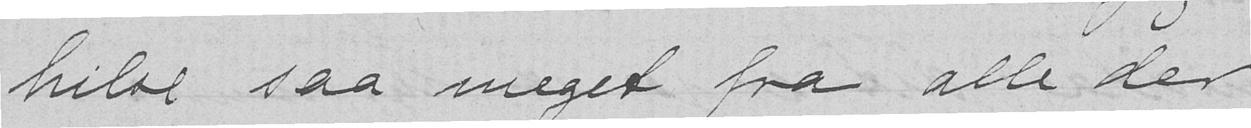hilse saa meget fra alle der
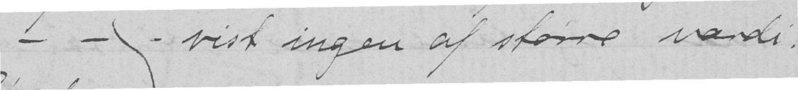- - - vist ingen af større værdi.
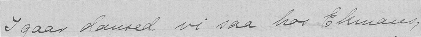I gaar dansed vi saa hos Ekmans,
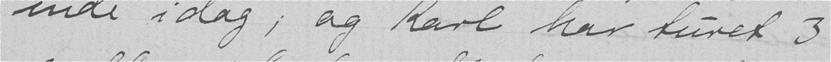inde idag; og Karl har turet 3
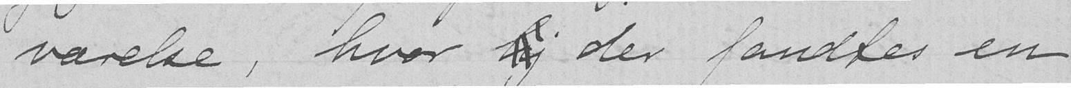værelse, hvor der fandtes en
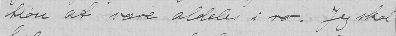tion at være aldeles i ro. Jeg skal
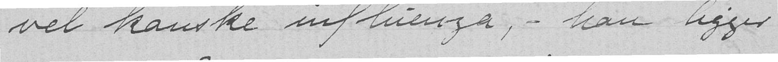vel kanske influenza, - han ligger
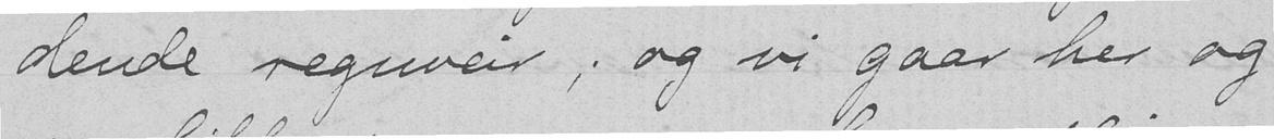dende regnveir, og vi gaar her og
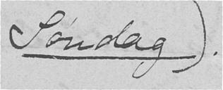Søndag.
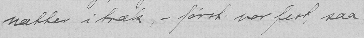nætter i stræk, - først vor fest, saa
